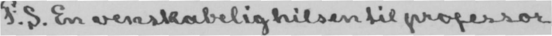P: S. En venskabelig hilsen til professor
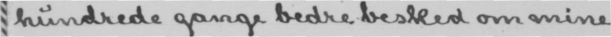hundrede gange bedre besked om mine
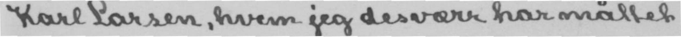Karl Larsen, hvem jeg desværr har måttet
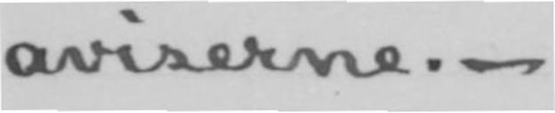aviserne.-
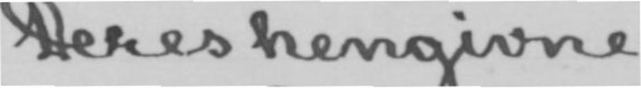Deres hengivne
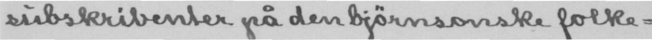subskribenter på den bjørnsonske folke-
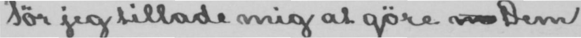Tør jeg tillade mig at gøre m Dem
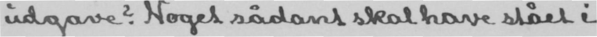udgave? Noget sådant skal have stået i
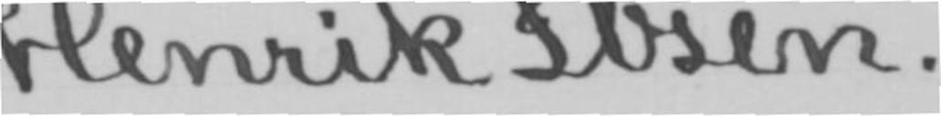Henrik Ibsen.
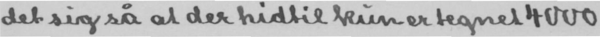det sig så at der hidtil kun er tegnet 4000
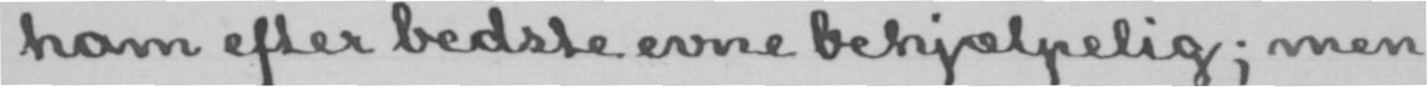ham efter bedste evne behjælpelig; men
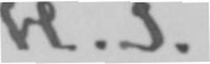H. I.
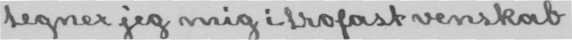tegner jeg mig i trofast venskab
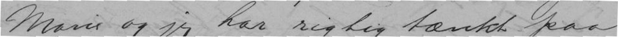Marie og jeg har rigtig tænkt paa
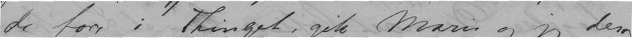de for i Thinget, gik Marie og jeg derop
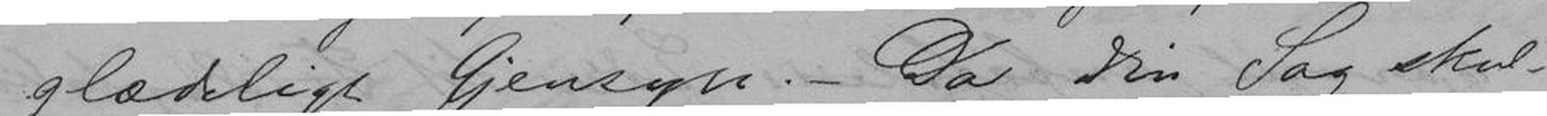glædeligt Gjensyn. - Da Din Sag skul-
